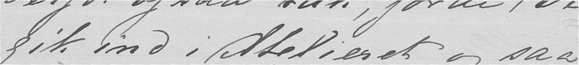gik ind i Atelieret, og saa
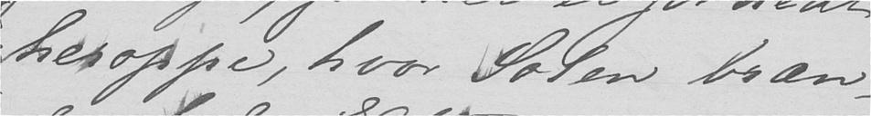heroppe, hvor Soten bræn-
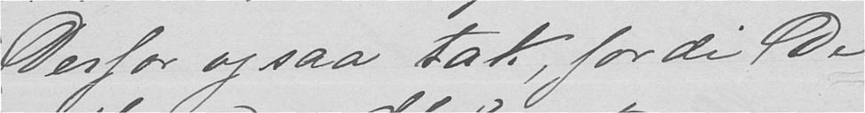Derfor ogsaa tak, fordi De
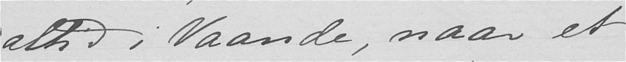altid i Vaande, naar et
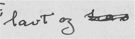lavt og has
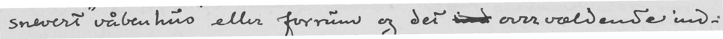snevert "våbenhus" eller forrum og det ind overvældende ind-
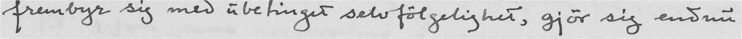frembyr sig med ubetinget selvfølgelighet, gjør sig endnu
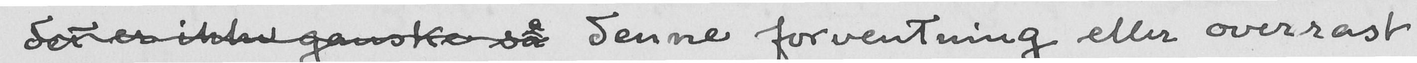det er ikke ganske så denne forventning eller overrask
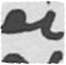et
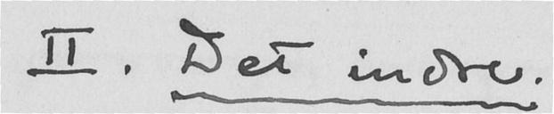II. Det indre.
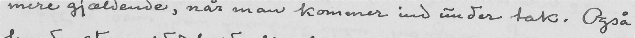mere gjældende, når man kommer ind under tak. Også
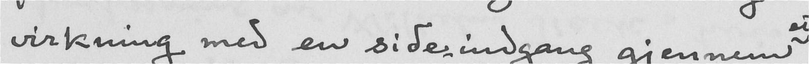virkning med en sideindgang gjennem
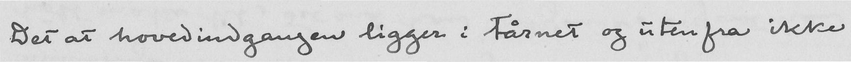Det at hovedindgangen ligger i tårnet og utenfra ikke
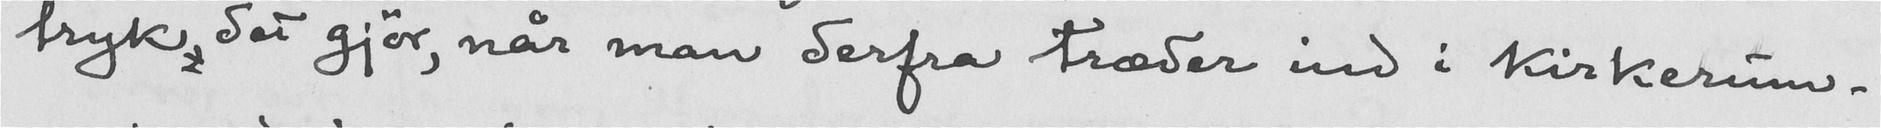tryk, det gjør, når man derfra træder ind i kirkerum-
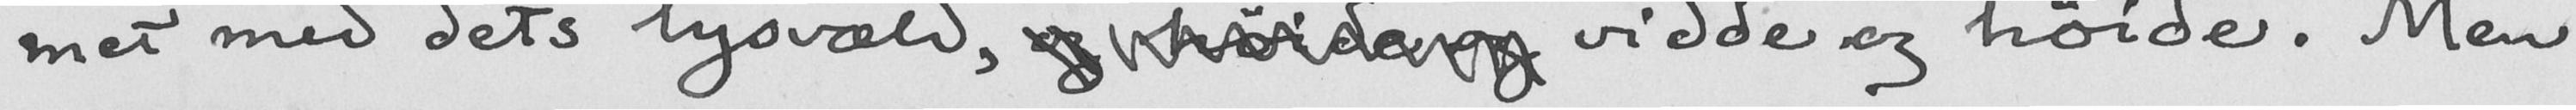met med dets lysvæld, og høide og vidde og høide. Men
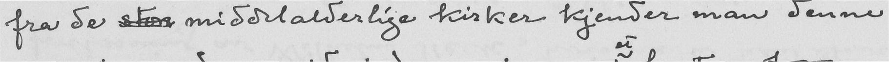fra de store middelalderlige kirker kjender man denne
